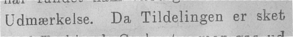Udmærkelse. Da Tildelingen er sket
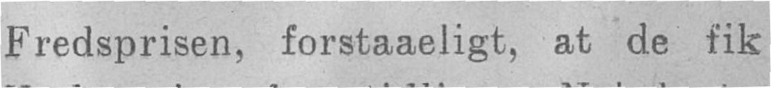Fredsprisen, forstaaeligt, at de fik
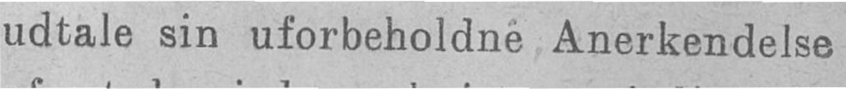udtale sin uforbenholdne Anerkendelse
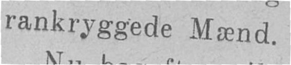rankryggede Mænd.
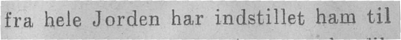fra hele Jorden har indstillet ham til
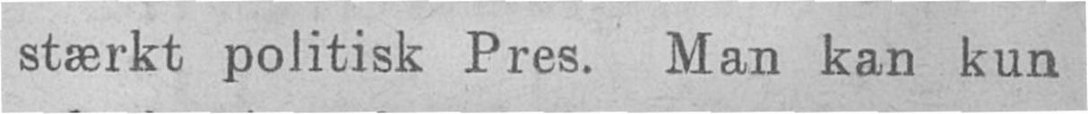stærkt politisk Pres. Man kan kun
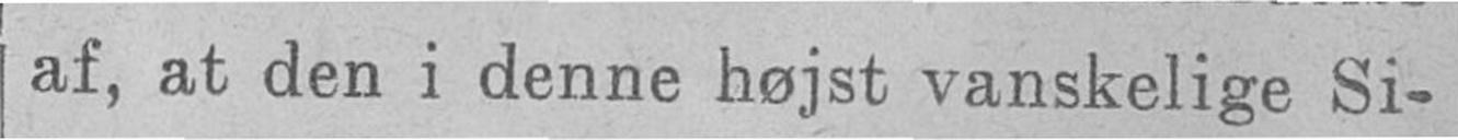af, at den i denne højst vanskelige Si-
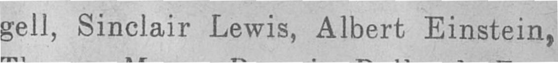gell, Sinclair Lewis, Albert Einstein,
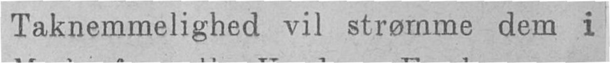Taknemmelighed vil strømme dem i
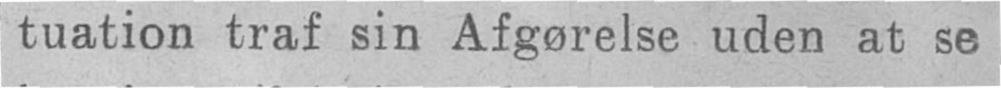tuation traf sin Afgørelse uden at se
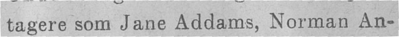tagere som Jane Addams, Norman An-
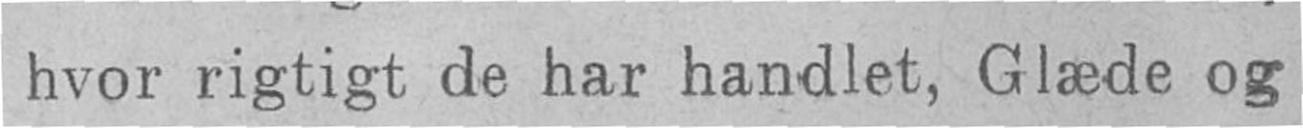hvor rigtig de har handlet, Glæde og
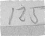125
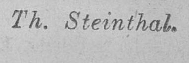Th. Steinthal.
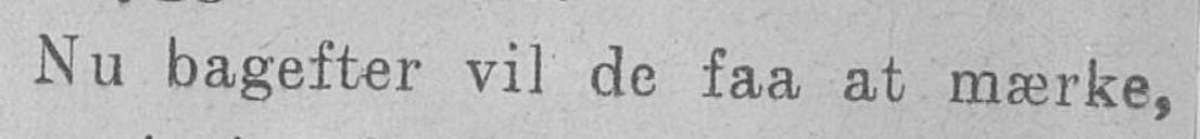Nu bagefter vil de faa at mærke,
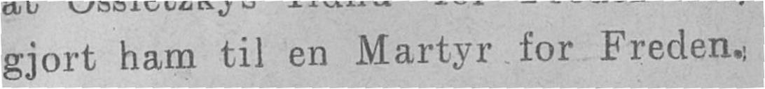gjort ham til en Martyr for Freden.
